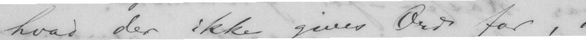hvad der ikke gives Ord for, og
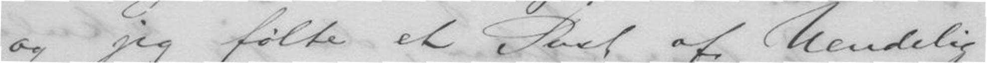og jeg følte et Pust af Uendelig-
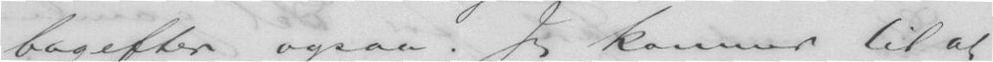bagefter ogsaa. Jeg kommer til at
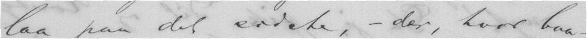laa paa det sidste, - der, hvor baa-
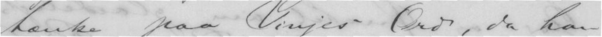tænke paa Vinjes Ord, da han
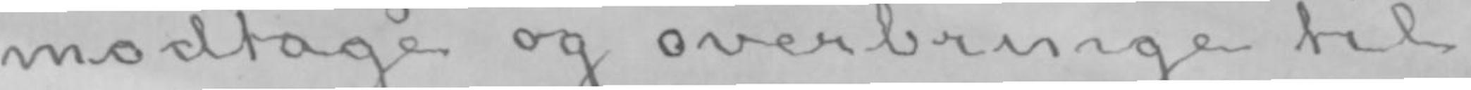modtage og overbringe til
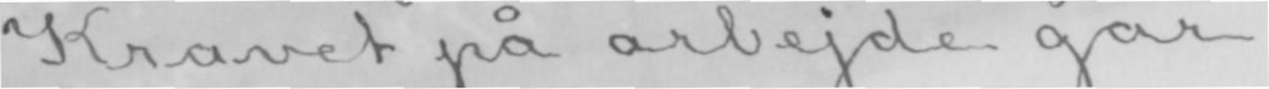Kravet på arbejde går
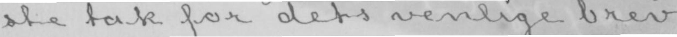ste tak for dets venlige brev
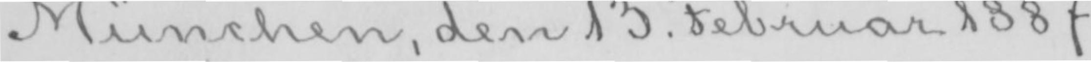München, den 13. Februar 1887.
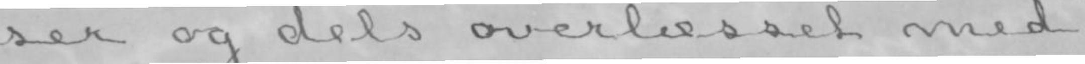ser og dels overlæsset med
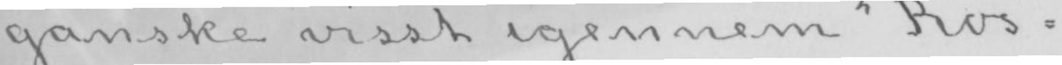ganske visst igennem «Ros-
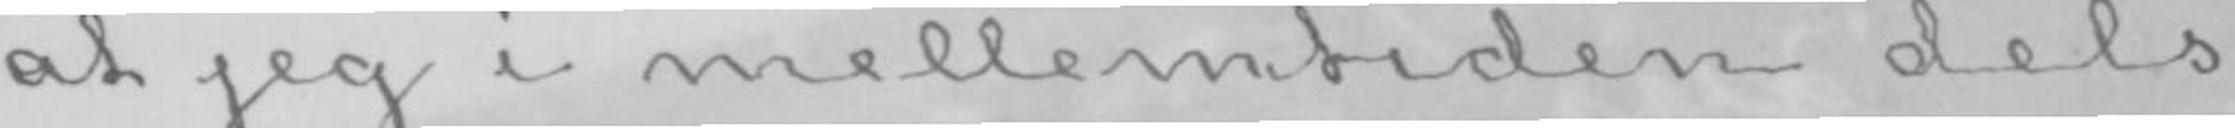at jeg i mellemtiden dels
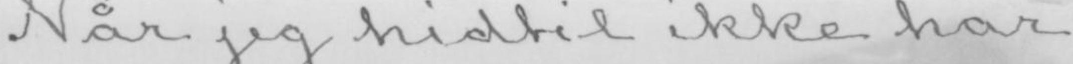Når jeg hidtil ikke har
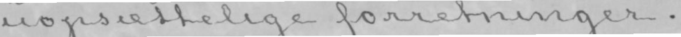uopsættelige forretninger.
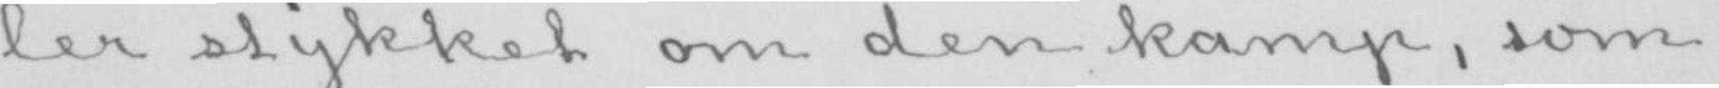ler stykket om den kamp, som
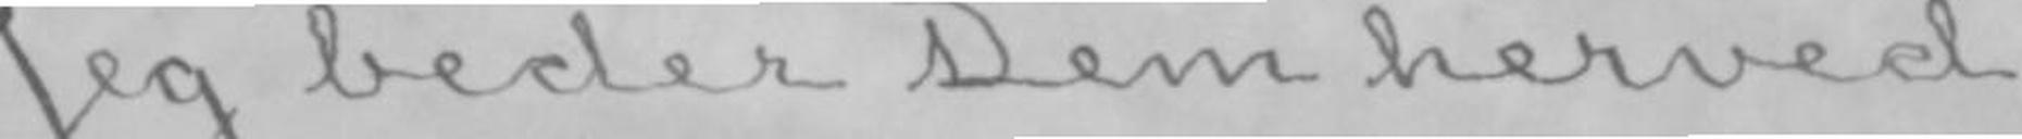Jeg beder Dem herved
